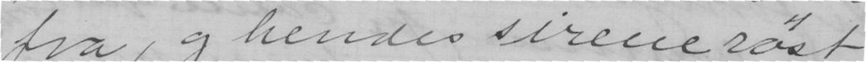fra, og hendes sirene röst
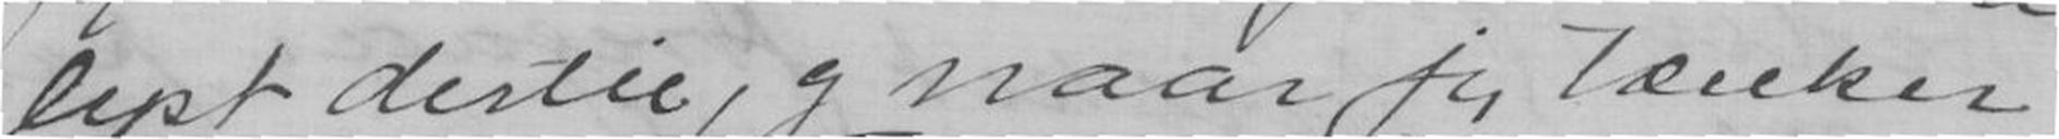lyst dertil, og naar jeg tænker
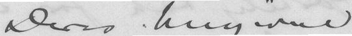Deres hengivne
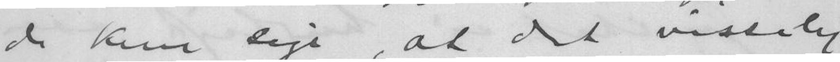de kun sige, at det visselig
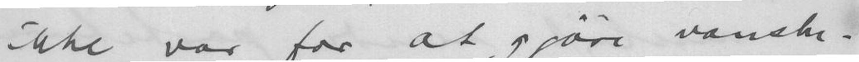ikke var for at gjøre vanske-
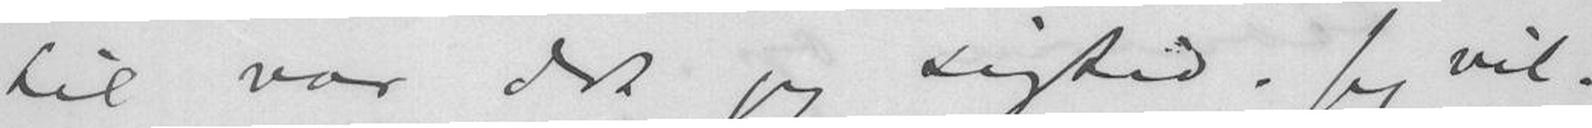til var det jeg sigted. Jeg vil-
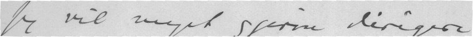Jeg vil meget gjerne dirigere
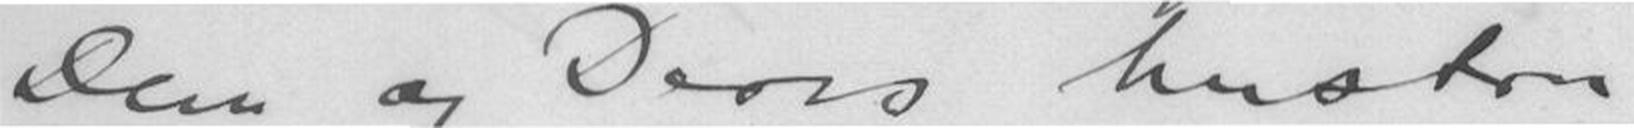Dem og Deres hustru
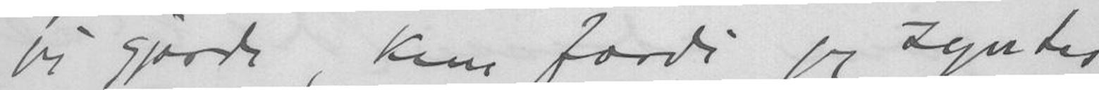jeg gjorde, kun fordi jeg syntes
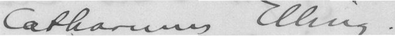Catharinus Elling
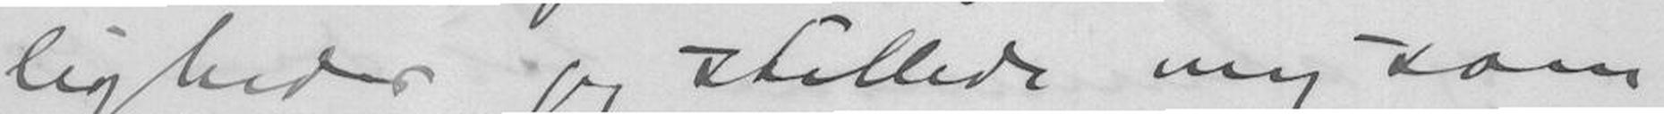ligheder jeg stillede mig som
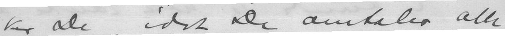ker De, idet De omtaler alle
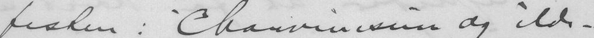festen: Chauvinisme og ilde-
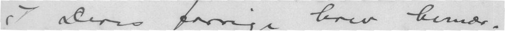I deres forrige brev bemær-
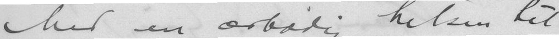Med en ærbødig hilsen til
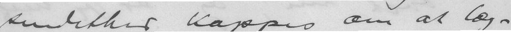sindethed kappes om at læg-
